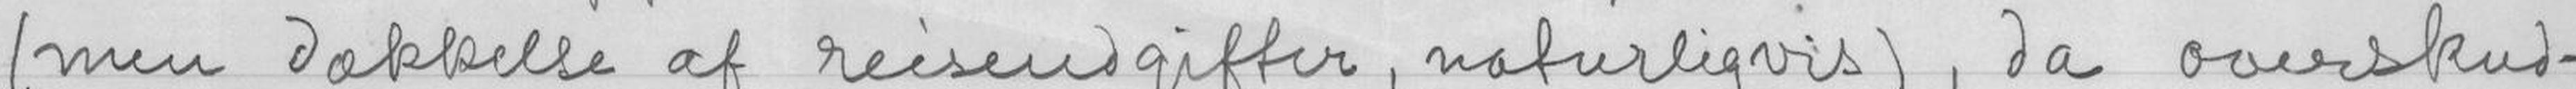(men dækkelse af reiseudgifter, naturligvis), da overskud-
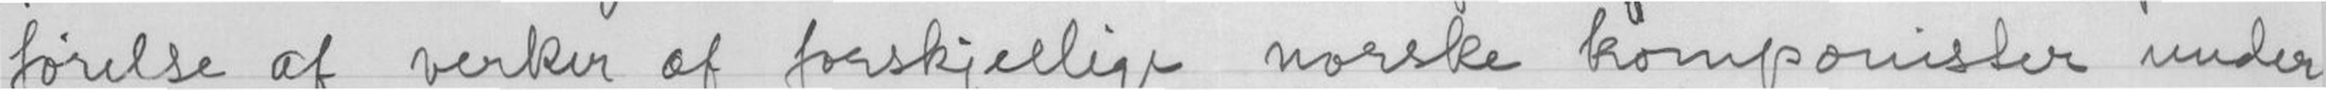førelse af verker af forskjellige norske komponister under
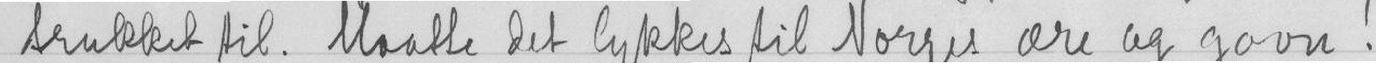trukket til. Maatte det lykkes til Norges ære og gavn!
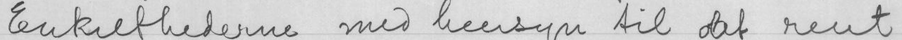Enkelthederne med hensyn til det rent
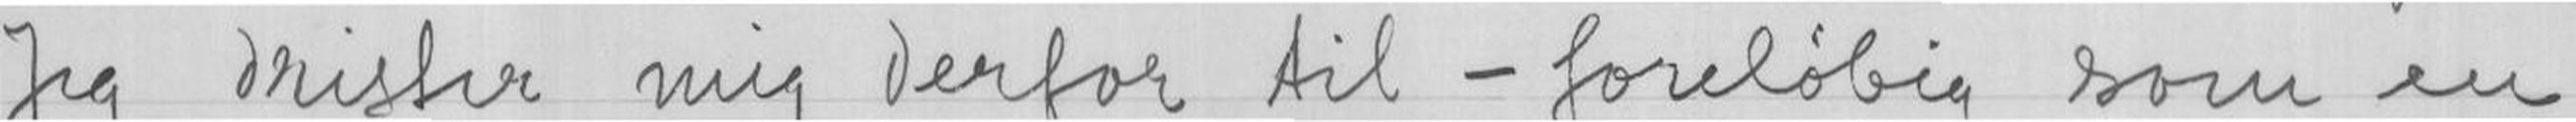Jeg drister mig derfor til - foreløbig som en
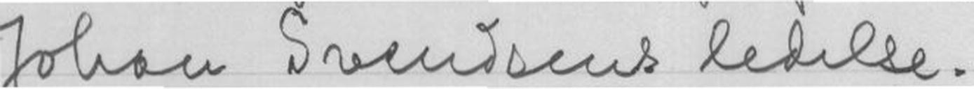Johan Svendsens ledelse.
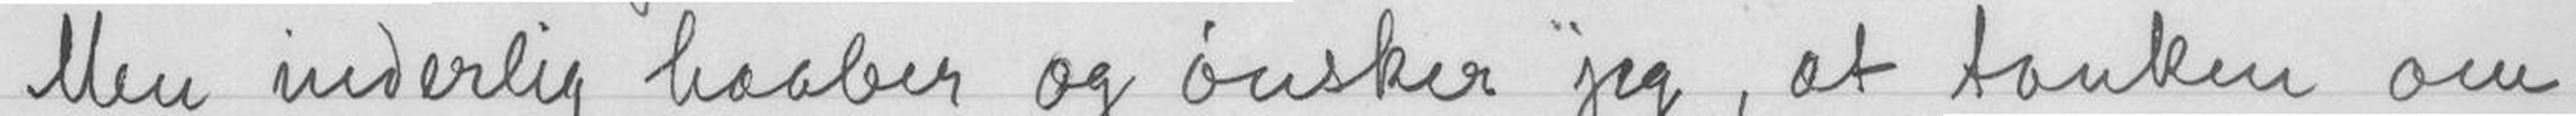Men inderlig haaber og ønsker jeg, at tanken om
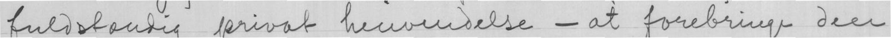fuldstændig privat henvendelse - at forebringe den
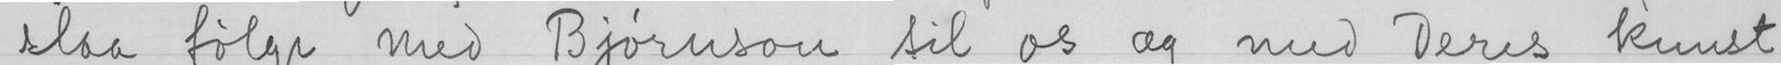slaa følge med Bjørnson til os og med Deres kunst
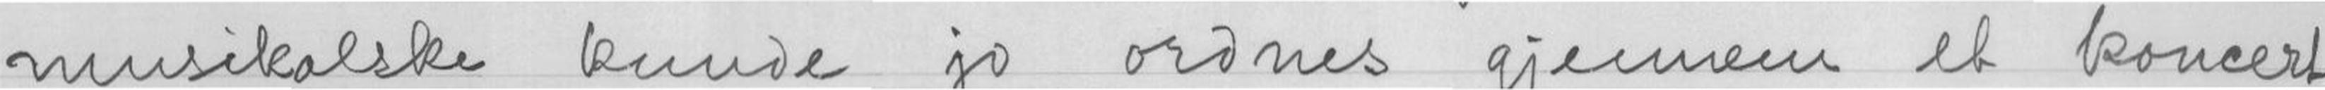musikalske kunde jo ordnes gjennem et koncert-
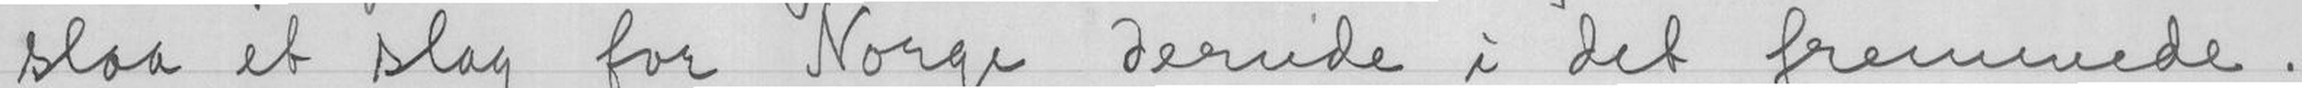slaa et slag for Norge derude i det fremmede.
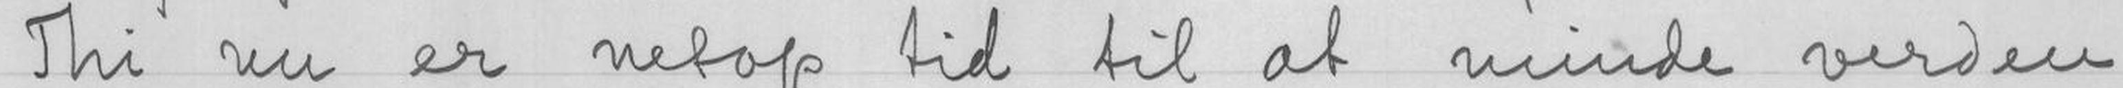Thi nu er netop tid til at minde verden
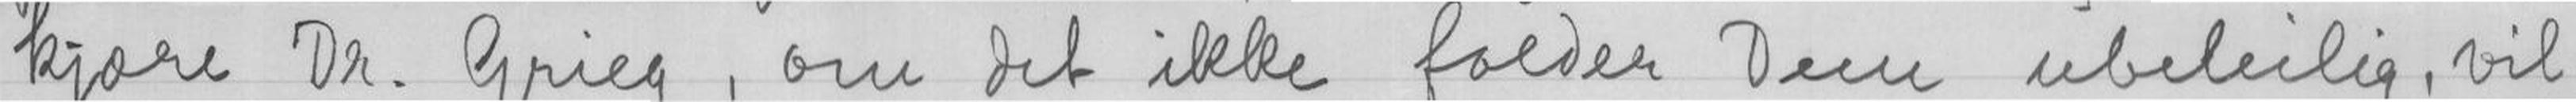kjære Dr. Grieg, om det ikke falder Dem ubeleilig, vil
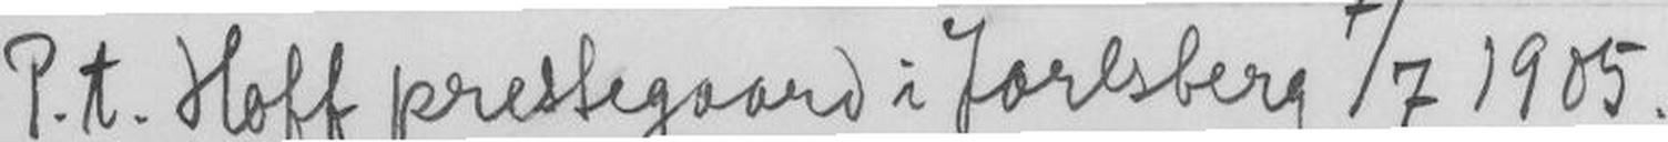P.t.Hoff prestegaard i Jarlsberg 7/7 1905.
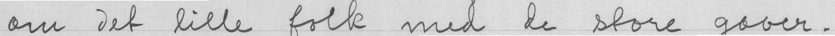om det lille folk med de store gaver.
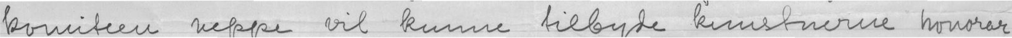komiten neppe vil kunne tilbyde kunstnerne honorar
